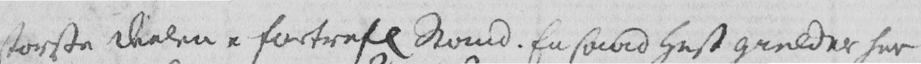første Delen i fortrefl Stand. En saad Hest gielder her
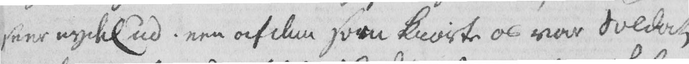seer nydel ud. een af dem som kiørte os var Soldat
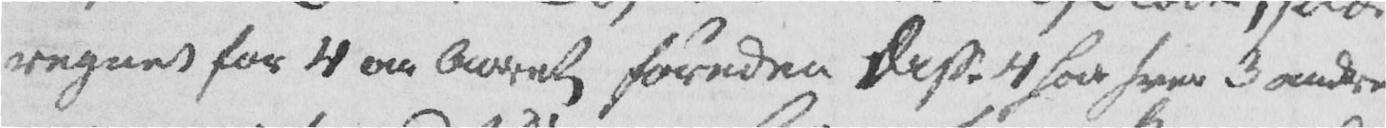regnet for 4 om Aaret foruden disse 4 han have 3 andre
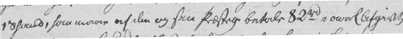1 Spand, han maae af den og sin Præsteg. betale 82 rd i aarl Afgivt
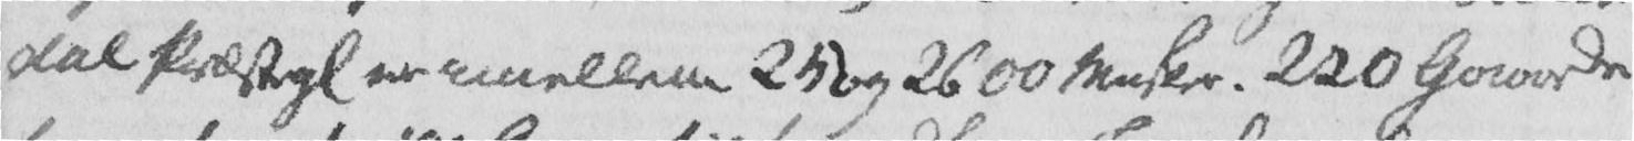dal Præstegl er imellem 25 og 2600 Mnskr. 220 Gaarde
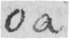0 a
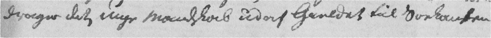drager det unge Mandskab udaf Gieldet til Søekanten
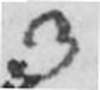3
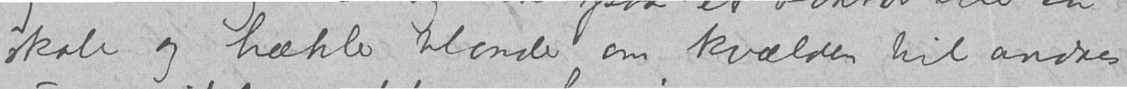skole og hækler blonder om kvælden til andres
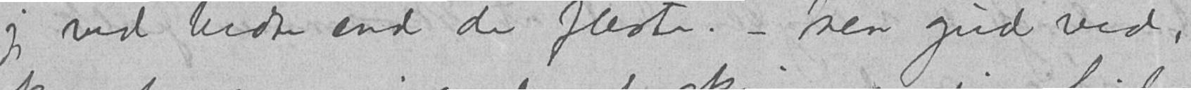jeg ved bedre end de fleste. - Men gud ved,
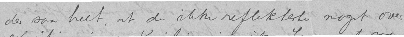der saa helt at de ikke reflekterte noget over
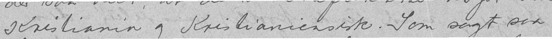Kristiania og Kristianiensisk. Som sagt saa
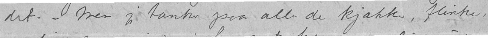det. - Men jeg tænker paa alle de kjække, flinke,
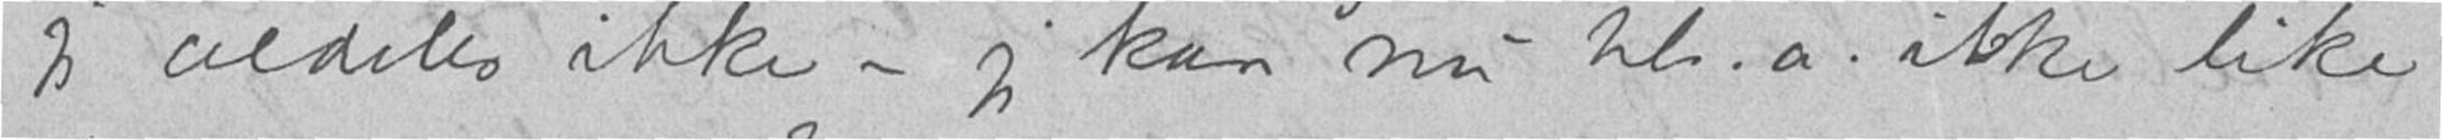jeg aldeles ikke - jeg kan nu bl.a. ikke like
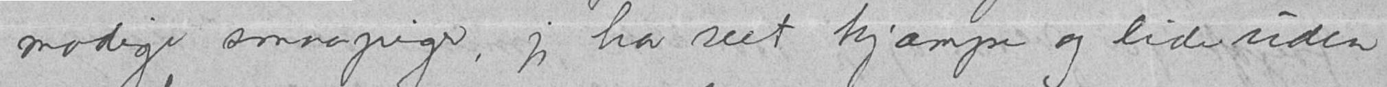modige smaapiger, jeg har seet kjæmpe og lide uden
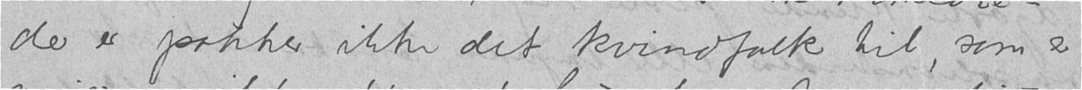der er pokker ikke det kvindfolk til, som er
